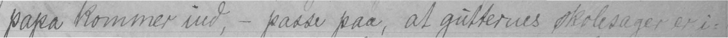papa kommer ind, - passe paa, at gutternes skolesager er i
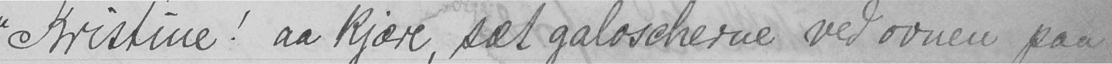"Kristine! aa kjære, sæt galoscherne ved ovnen paa
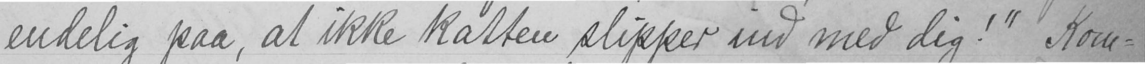endelig paa, at ikke katten slipper ind med dig!" Kom-
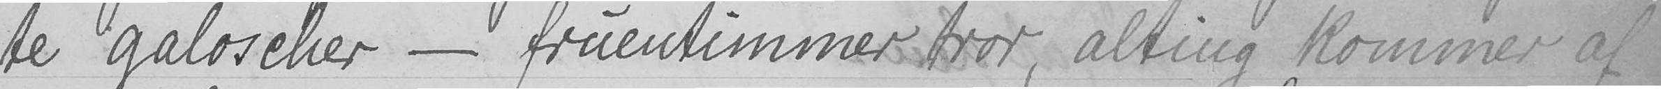te galoscher - fruentimmer tror, alting kommer af
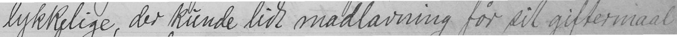lykkelige, der kunde lidt madlavning før sit giftermaal
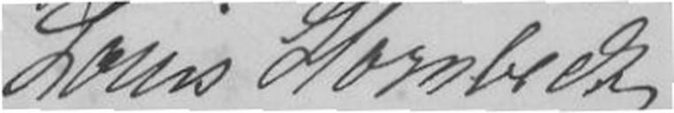Louis Hornbeck
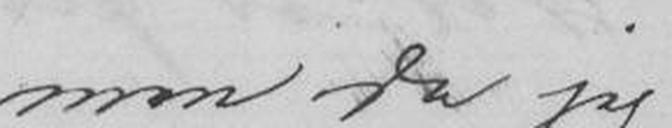men da jeg
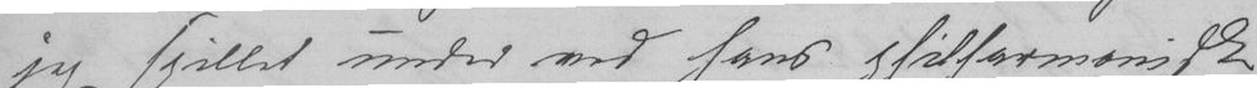jeg spillet under ved hans philharmoniske
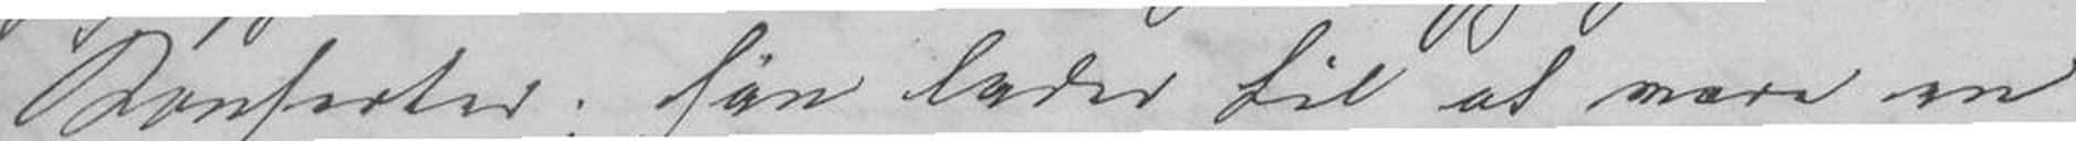konserter; han lader til at være en
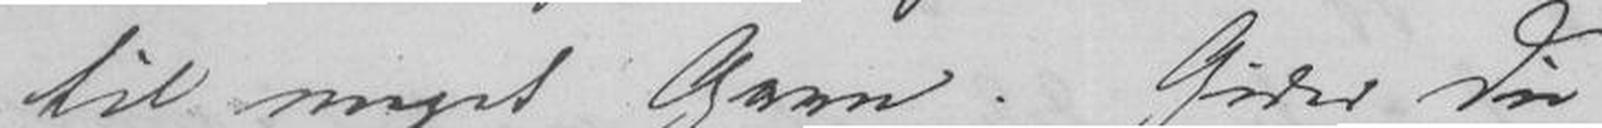til meget Gavn. Gidder Du
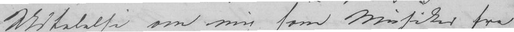Udtalelse om meg som musiker fra
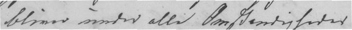bliver under alle Omstændigheder
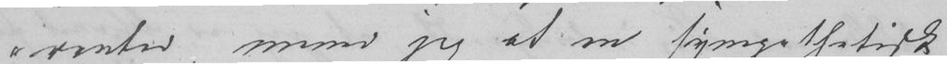renter, men jeg at en sympathisk
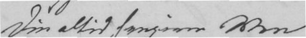Din altid hengivne Ven
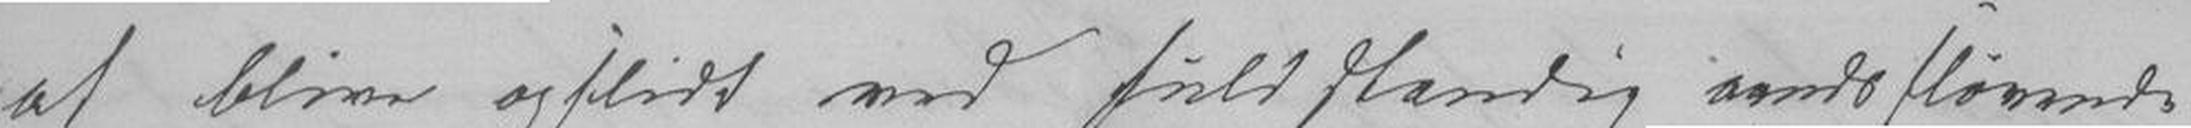at blive opslidt ved fuldstændig Aandssløvende
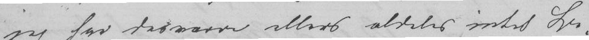jeg har desværre ellers aldeles intet Be-
